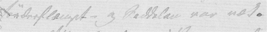sønderflænget – og Seddelen var væk.
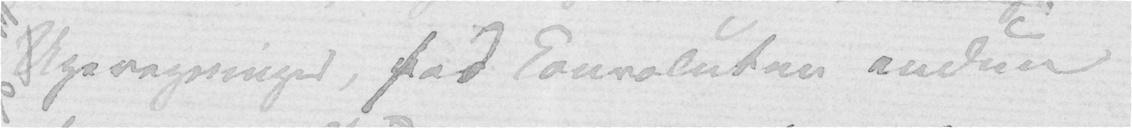Ugeregninger, sad Konvoluten endnu
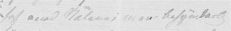fast ened Nålene, men besynderlig
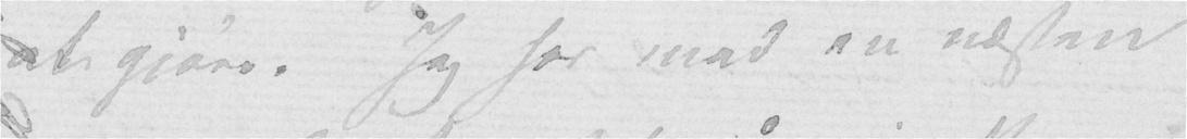at gjøre. Jeg har med en næsten
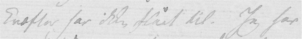Kræfter har ikke slået til. Jeg har
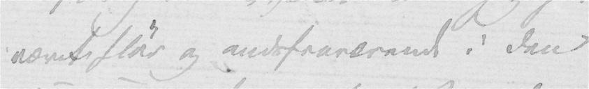været sløv og andsfraværende i den
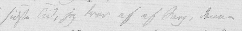sidste Tid, jeg tror af af Sorg, denne
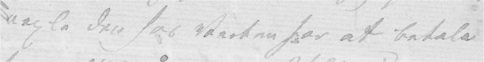vexle den hos Verten for at betale
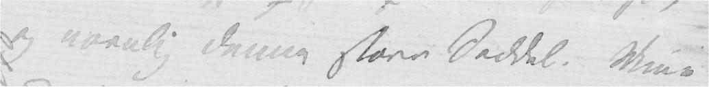og navnlig denne store Saddel. Mine
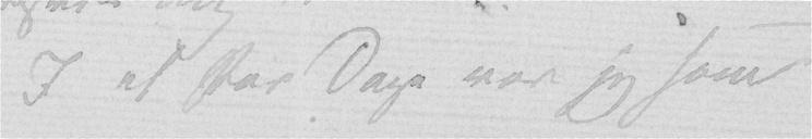I et Par Dage var jeg som
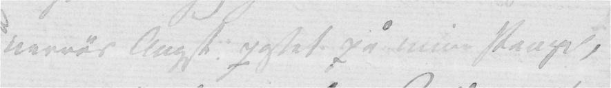nervøs Angst passet på mine Penge,
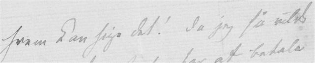hvem kan sige det! Da jeg så vilde
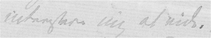interessere mig at vide.
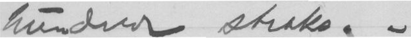hudrede straks. -
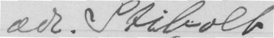adr. Stibolt
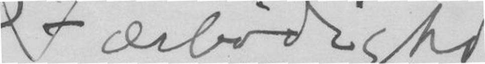I ærbødighed
